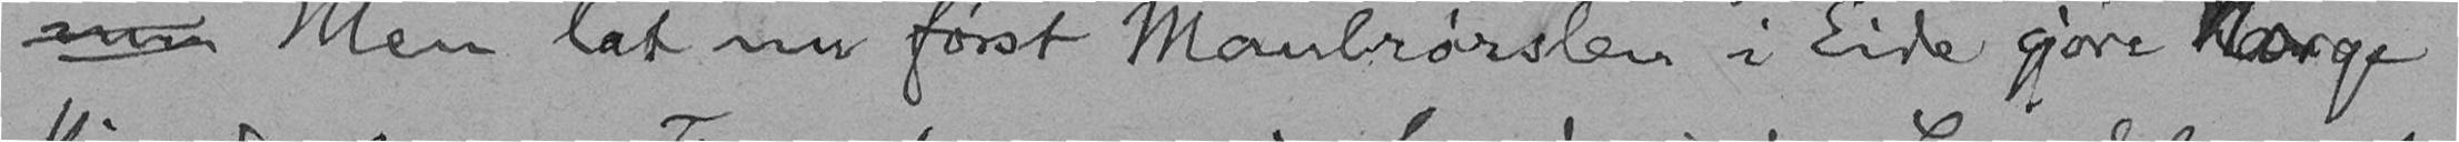men Men lat nu først Maulrørslen i Eide gjøre Norge
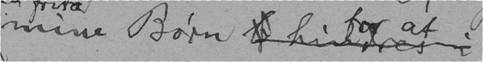mine Børn b hindres i
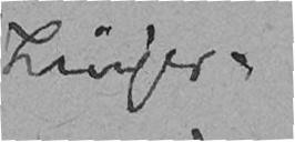Linjer.
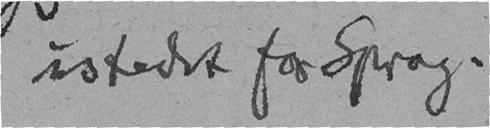istedet for Sprog.
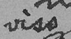viss
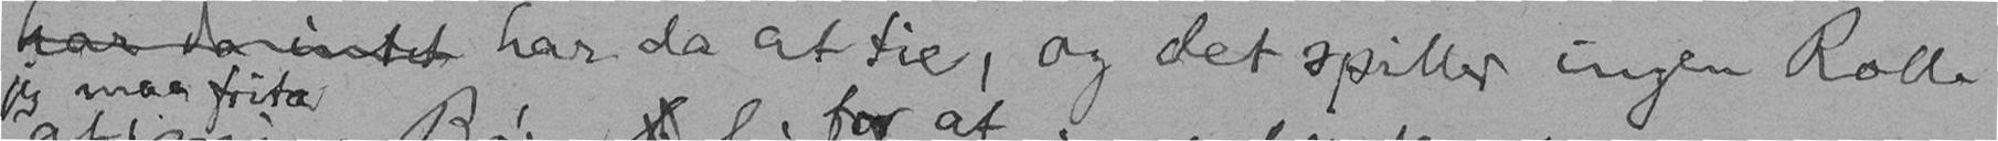har da intet har da at tie, og det spiller ingen Rolle
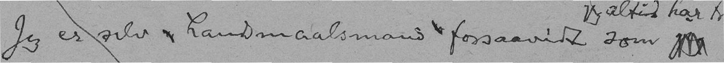Jeg er selv "Landsmaalsmand" forsaavidt som jeg
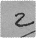2
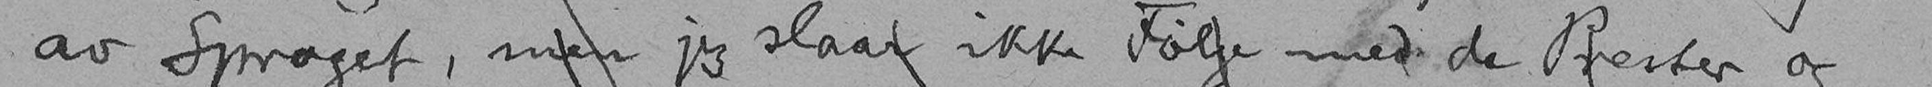av Sproget, men jeg slaar ikke Følge med de Prester og
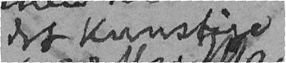det kunstige
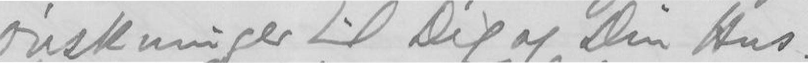ønsninger til Dig og Din Hus-
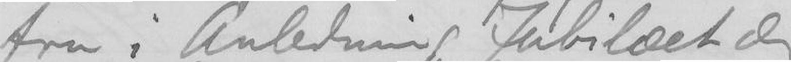tru i Anledning Jubelæet og
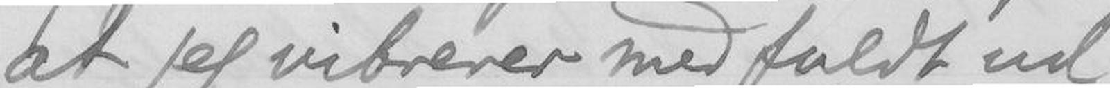at jeg vibrerer med fuldt ud -
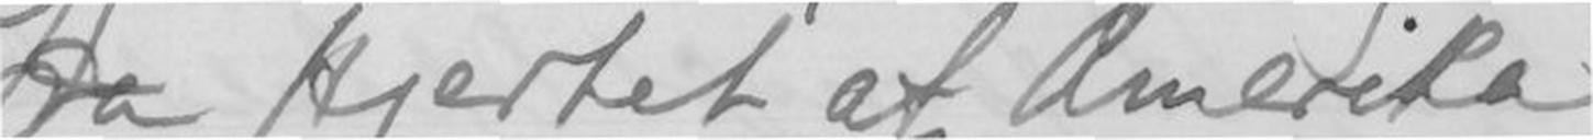Fra I Hjertet af Amerika
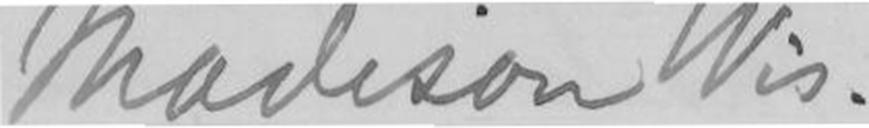Madison Wis.
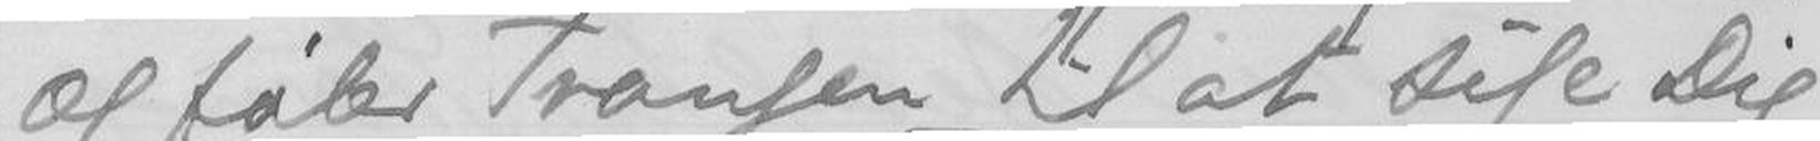og føler Trangen til at sige Dig
